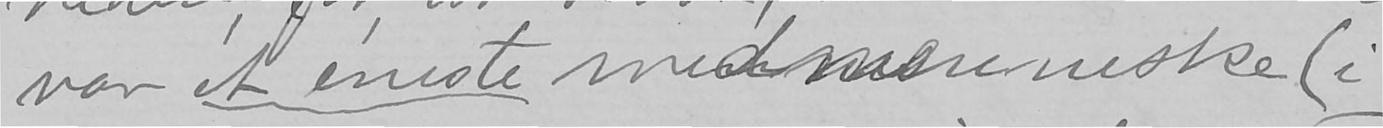var ét éneste medmenneske (i
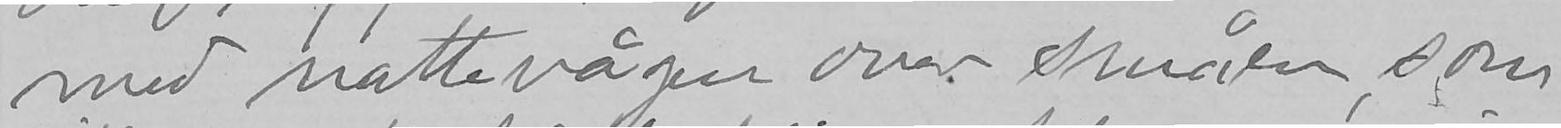med nattevågen over Småen, som
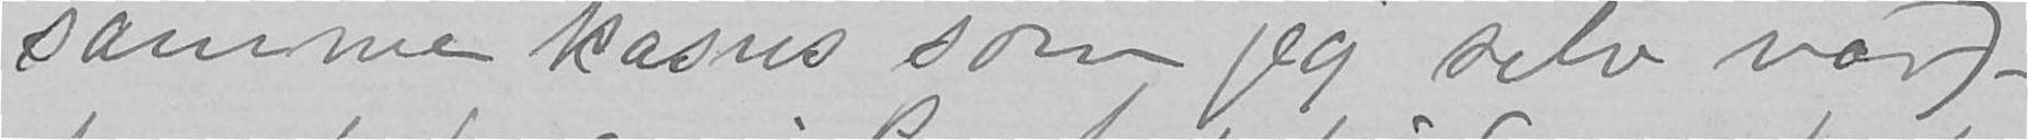samme kasus som jeg selv var) -
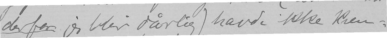derfor jeg blir dårlig) havde ikke kun-
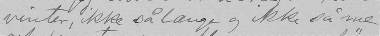vinter, ikke så længe og ikke så me-
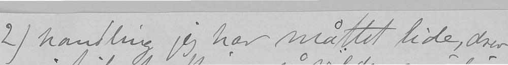2) handling jeg har måttet lide, drev
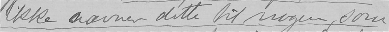ikke nævner dette til nogen, som
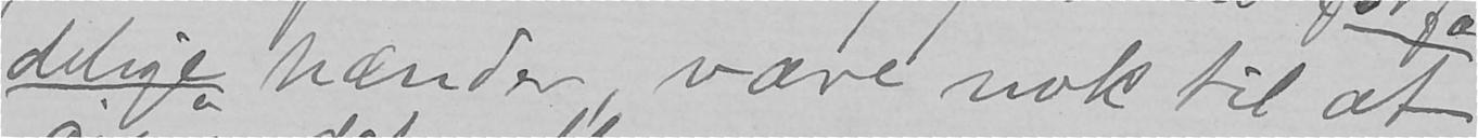delige hænder, være nok til at
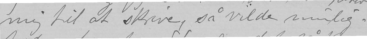mig til at skrive, så vilde mulig-
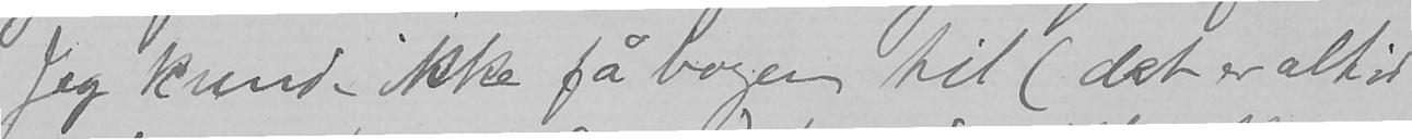Jeg kunde ikke få bogen til (det er altid
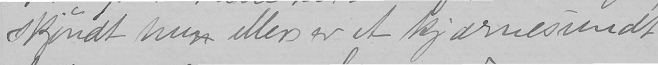skjøndt hun ellers er et kjærnesundt
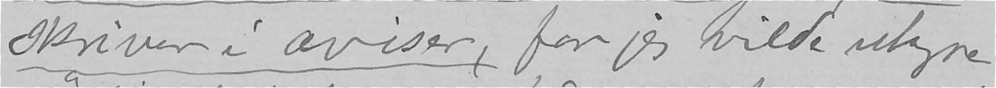skriver i aviser, for jeg vilde uhyre
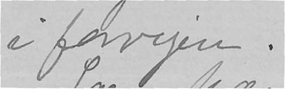i forvejen.
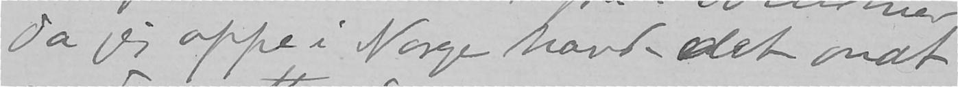da jeg oppe i Norge havde det ondt
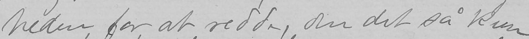heden, for at redde, om det så kun
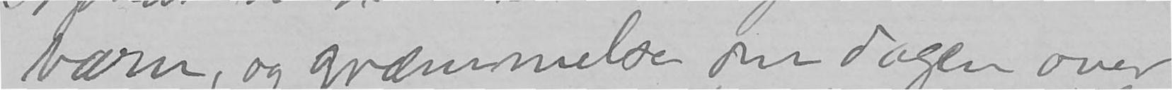barn, og græmmelse om dagen over
